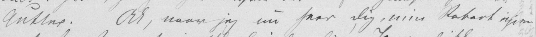Gutter. Ak, naar jeg nu seer Dig, min Robert igjen
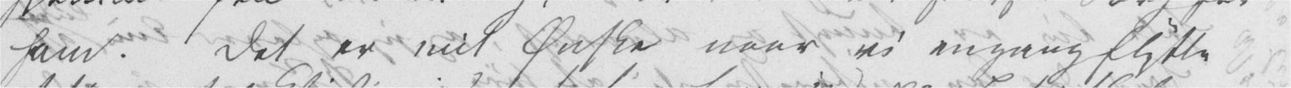ham. Det er mit Ønske naar vi engang flytte
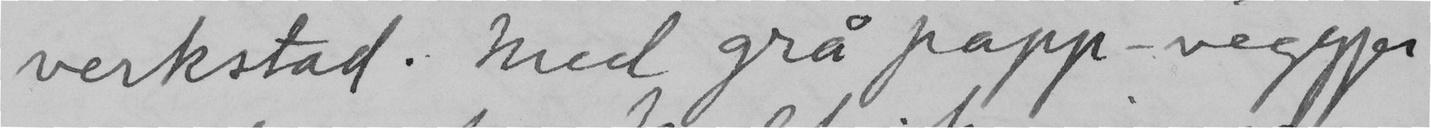verkstad. Med grå papp-veggjer
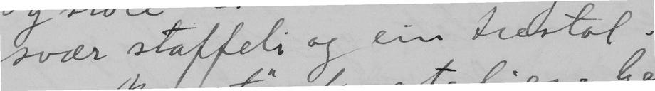svær staffeli og ein trestol.
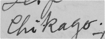Chikago.
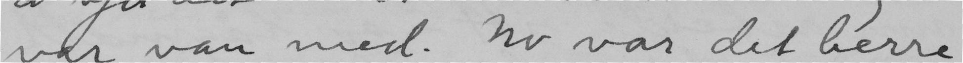var van med. No var det berre
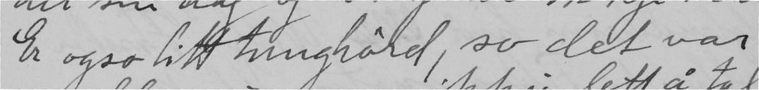Er ogso litt tunghörd, so det var
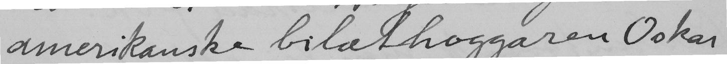amerikanske bilæthoggaren Oskar
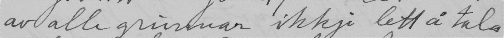av alle grunnar ikkje lett å tala
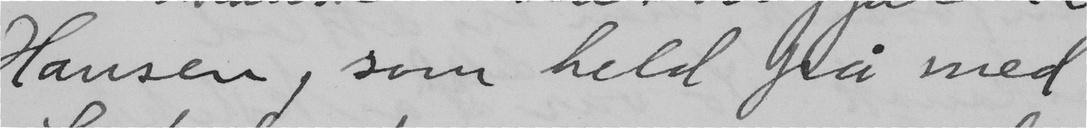Hansen, som held på med
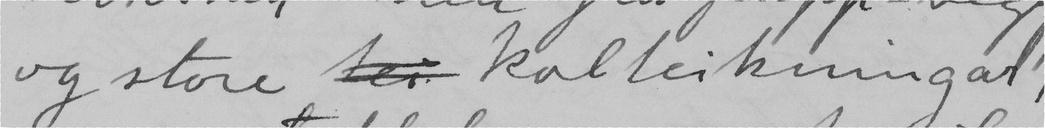og store  kolteikningar,
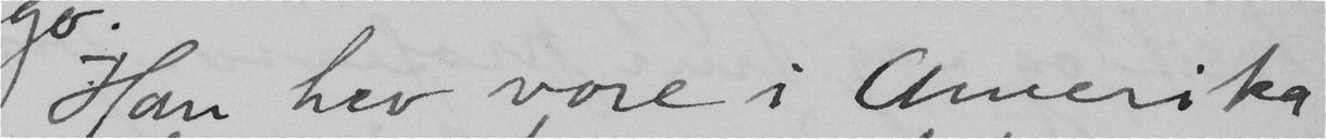Han hev vore i Amerika
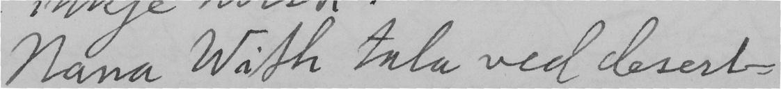Nana With tala ved desert-
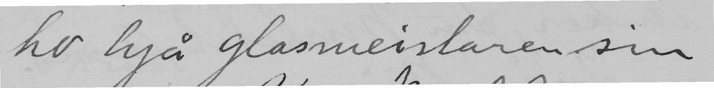ho hjå Glasmeistaren sin –
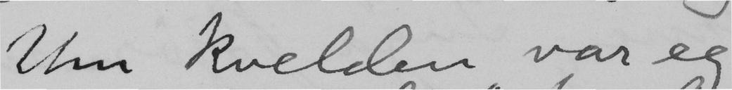Um kvelden var eg
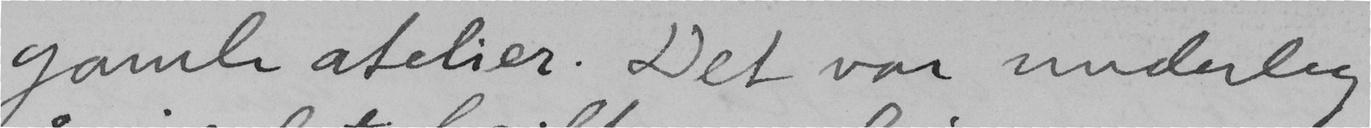gamle atelier. Det var underleg
